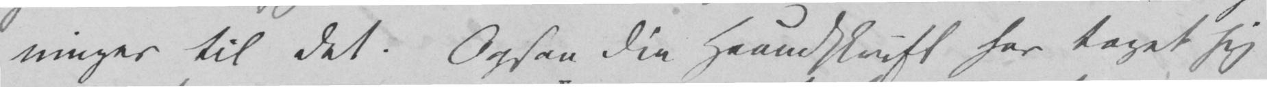ninger til det. Ogsaa Din Haandskrift har taget sig
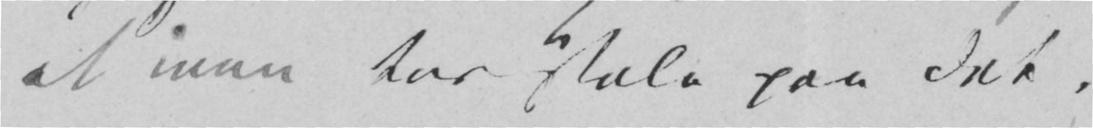at man tør stole paa det.
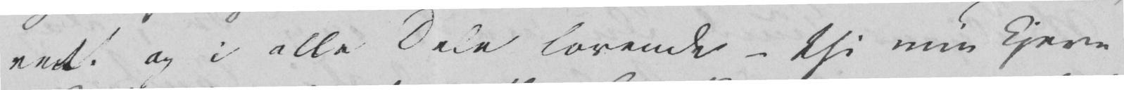vet! og i alle Dele lovende – thi min kjere
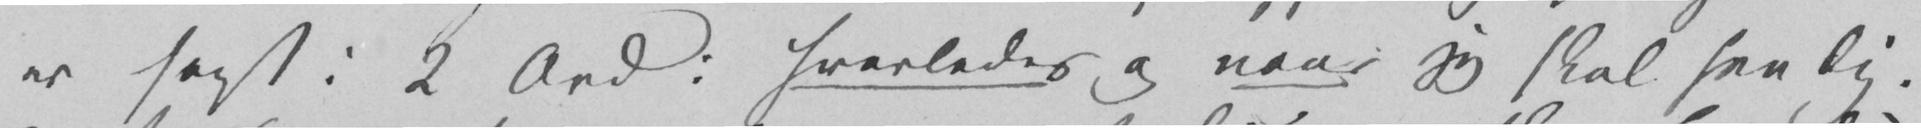er sagt i 2 Ord: hvorledes og naar jeg skal see Dig.
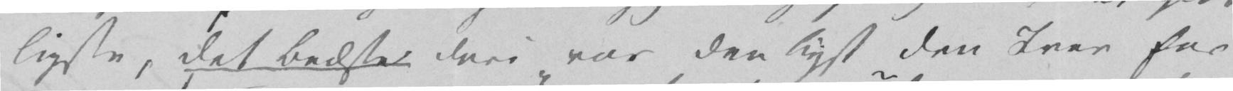ligste, det Bedste deri var den Lyst den Iver for
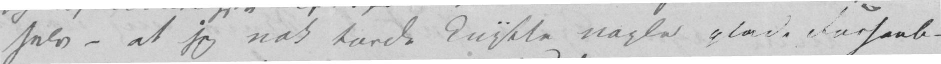selv – at jeg nok torde knytte nogle glade Forhaab-
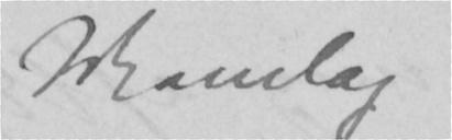Mandag.
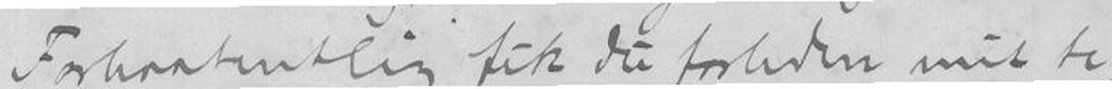Forhaabentlig fik du forleden mit te
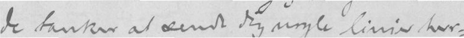de tanker at sende dig nogle linier her-
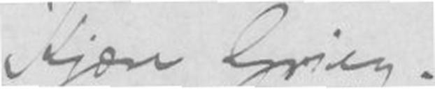Kjære Grieg!
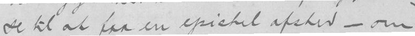se til at faa en epistel afted - om
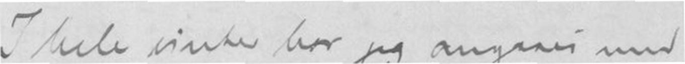I hele vinter har jeg omgaaes med
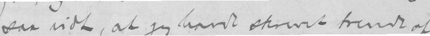saa vidt, at jeg havde skrevet tvende af
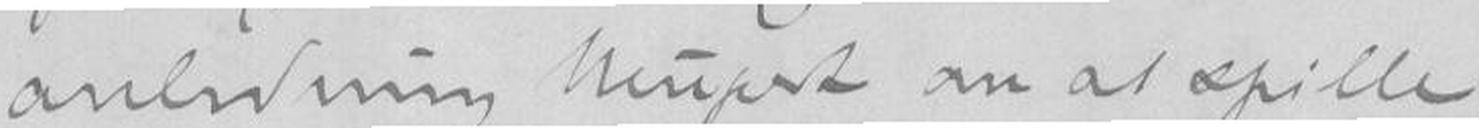anledning Neupert om at spille
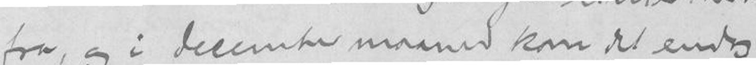fra, og i december maaned kom det endog
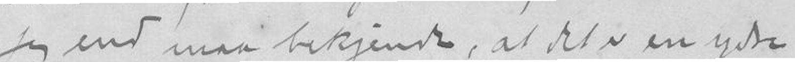jeg end maa bekjende, at det i en ydtre
